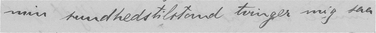min sundhedstilstand tvinger mig saa
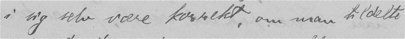i sig selv være korrekt, om man tildelte
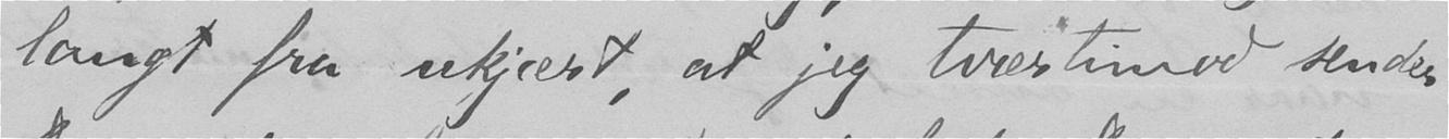langt fra ukjært, at jeg tværtimod sender
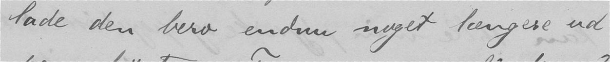lade den bero endnu noget længere ud
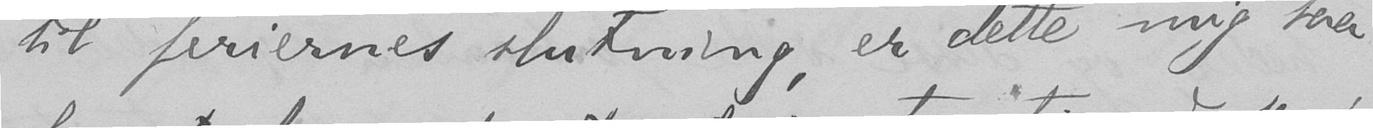til feriernes slutning, er dette mig saa
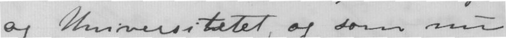og Universitetet, og som nu
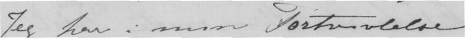Jeg har i min Fortvivlelse
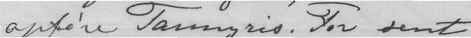opføre Tamyris. For sent
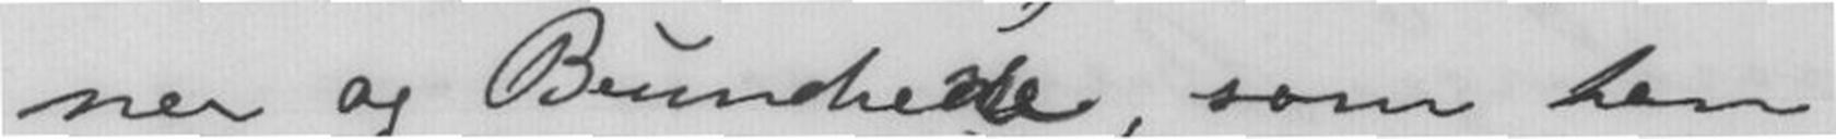ner og Beundrere, som han
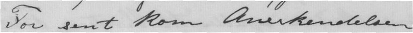For sent kom Anerkendelsen
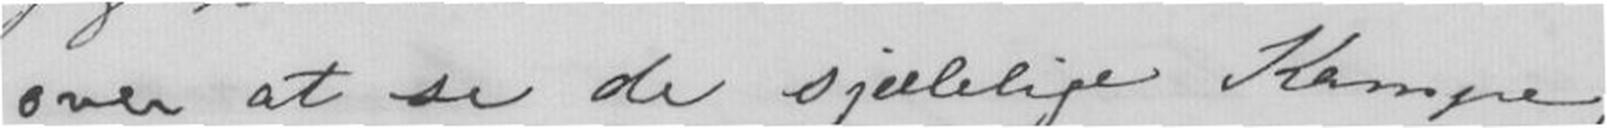over at se de sjælelige Kamper,
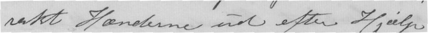rakt Hænderne ud efter Hjælp
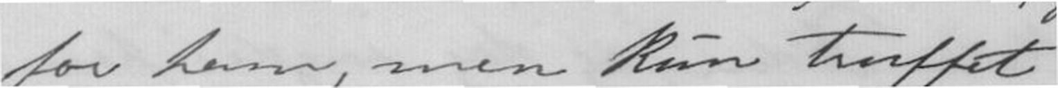for ham, men kun truffet
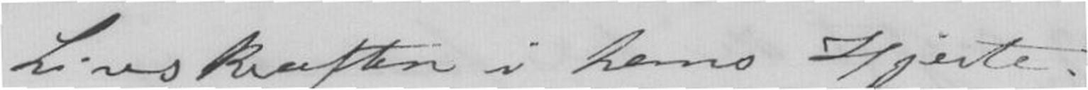Livskraften i hans Hjerte.
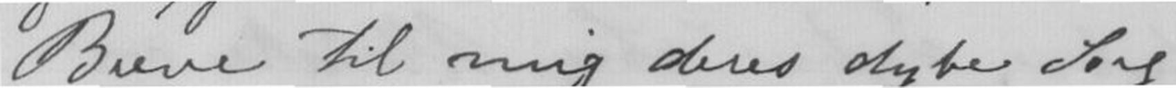Breve til mig deres dybe Sorg
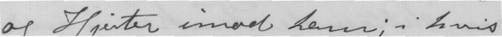og Hjerter imod ham; i hvis
